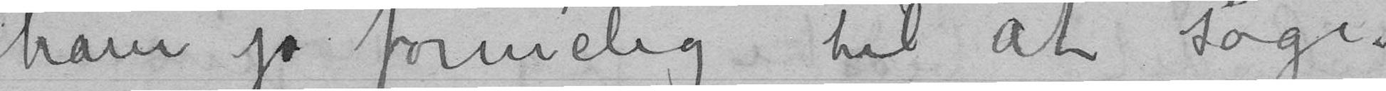ham jo formelig til at søge.
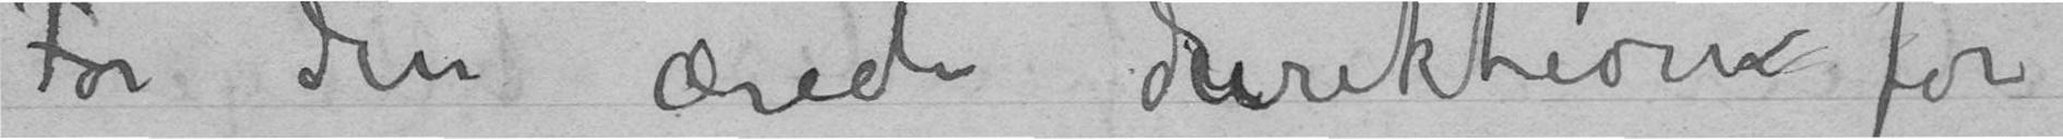For den ærede direktion for
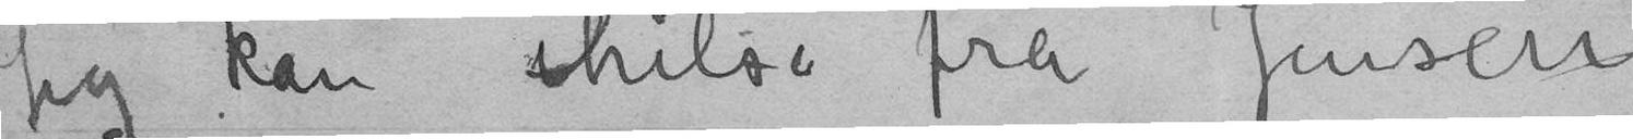Jeg kan hilse dig fra Jensen
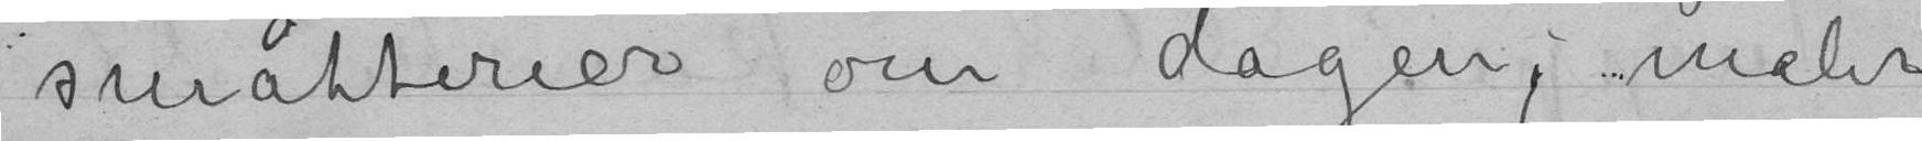småtterier om dagen, maler
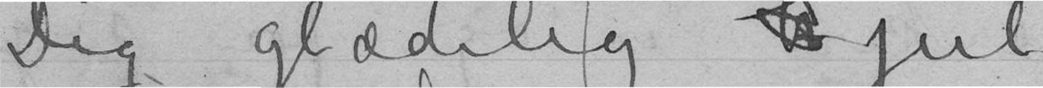Dig glædelig h jul.
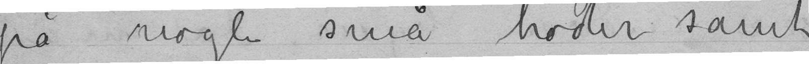på nogle små hoder samt
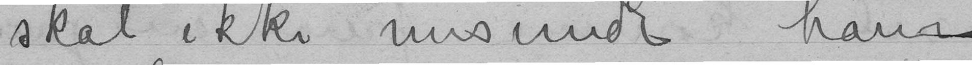skal ikke misunde ham
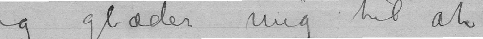Jeg glæder mig til at
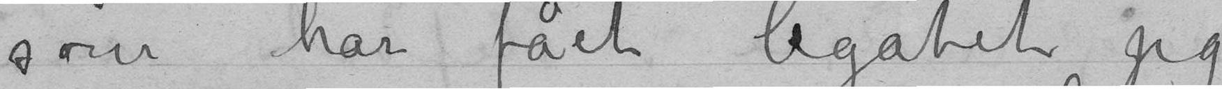som har faet legatet jeg
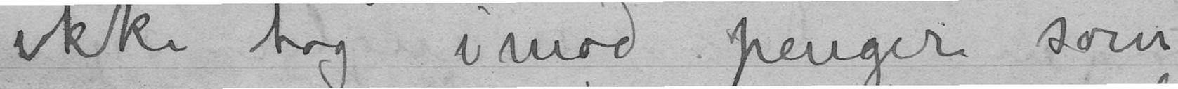ikke tog imod penger som
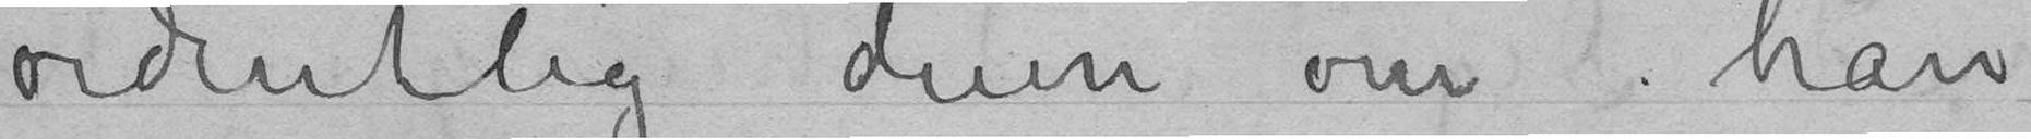ordentlig dum om han
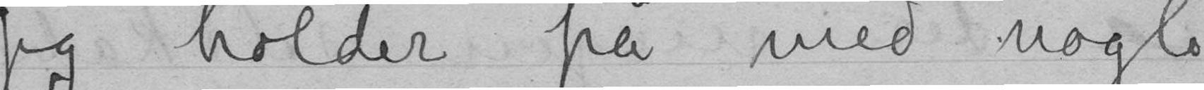Jeg holder på med nogle
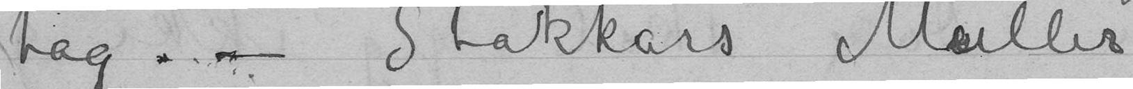tag. – Stakkars Müller
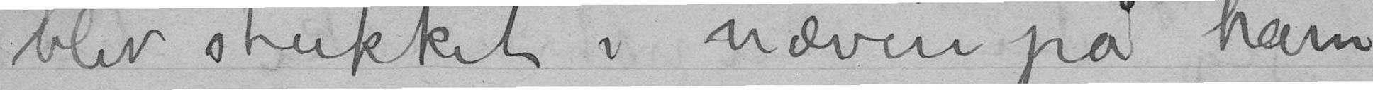blir stukket i næven på ham
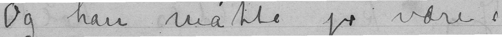Og han måtte jo være
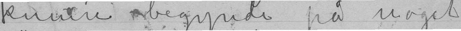kunne begynde på noget
